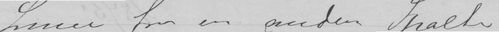Linie fra en anden Spalte
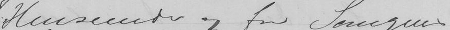Henseende og fra Sangens
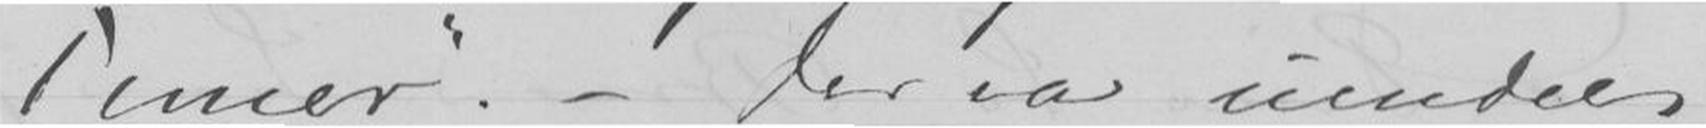Timer". - Der var uendelig
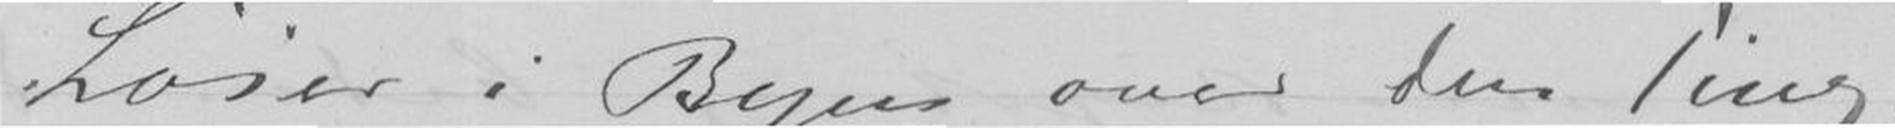Løier i Byen over den Ting.
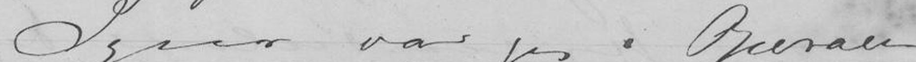Igaar var jeg i Operaen
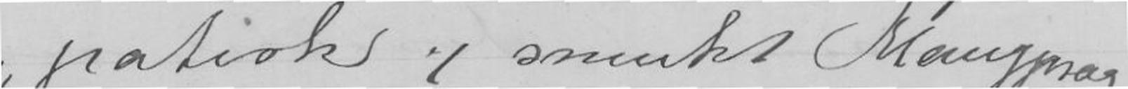patisk og smukt Klangpræg.
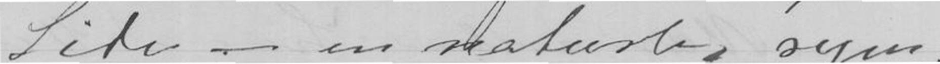Side - en naturlig sym-
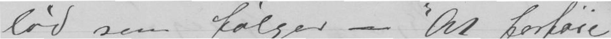lød som følger - "At forføie
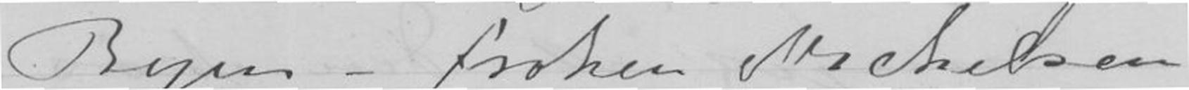Byen - frøken Michelsen
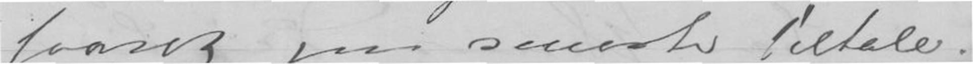svaret paa seneste Tiltale.
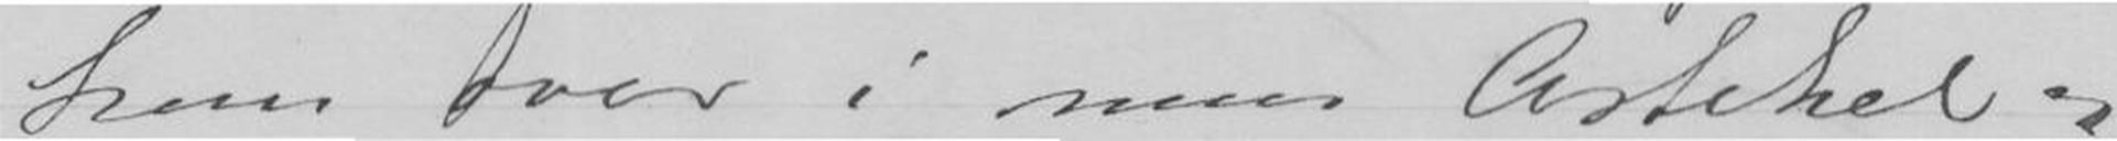kom over i min Artikel og
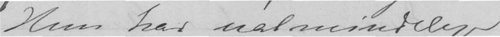Hun har ualmindelige
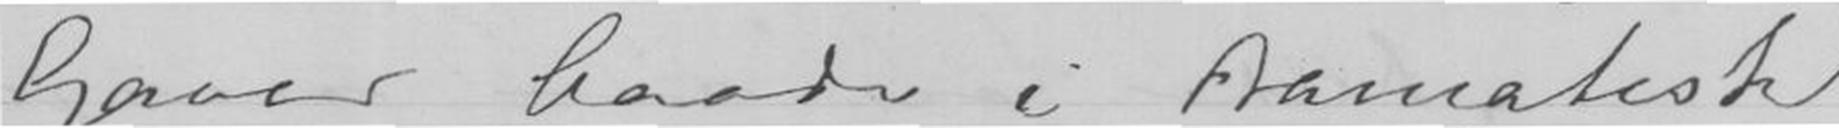Gaver baade i dramatisk
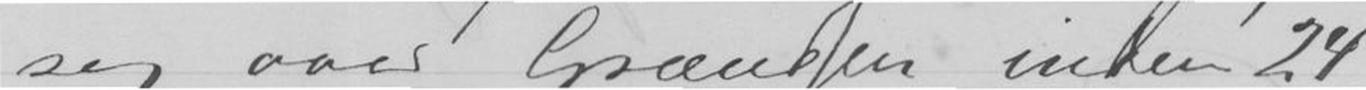sig over Grændsen inden 24
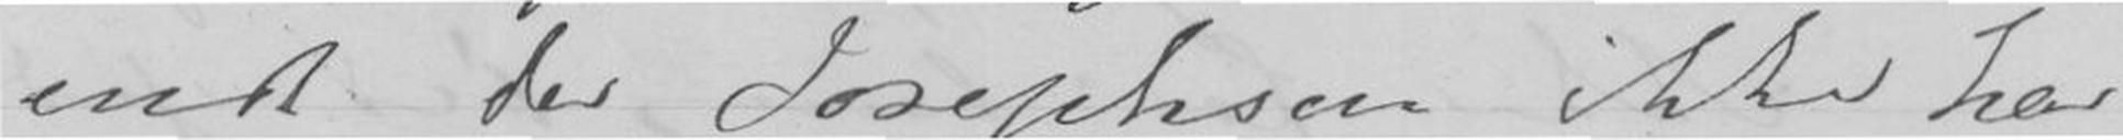endt da Josephson ikke har
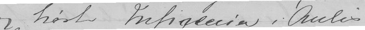og hørte Infigenia i Aulis
